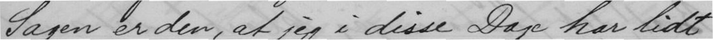Sagen er den, at jeg i disse Dage har lidt
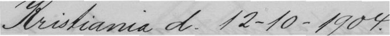Kristiania d. 12-10-1904.
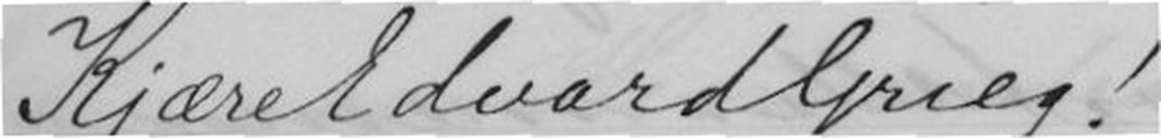Kjære Edvard Grieg!
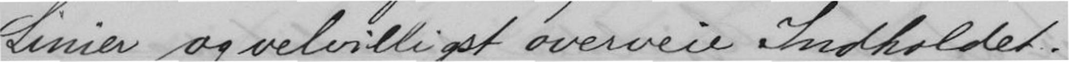Linier og velvilligst overveie Indholdet.
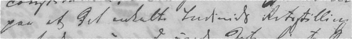paa at det enkelte Individs Retsstilling
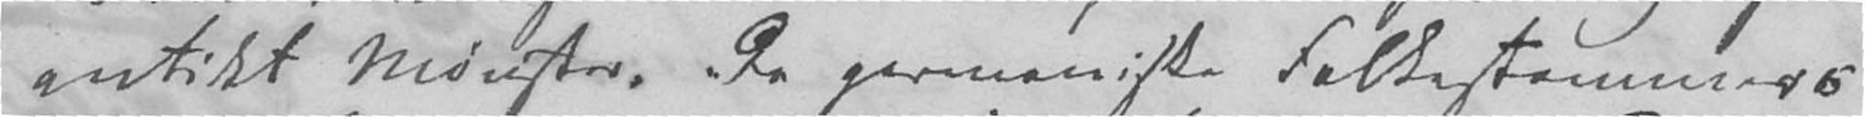antikt Mønster. De germaniske Folkestammers
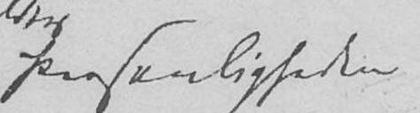Personligheden
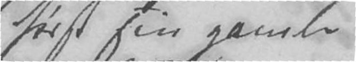først sin gamle
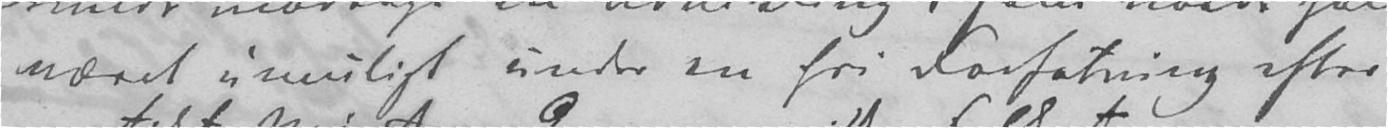været umuligt under en fri Forfatning efter
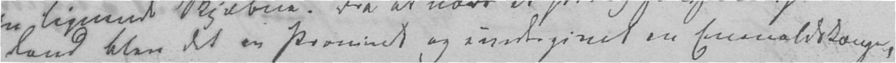Land blev det en Provinds og undergivet en Enevolds,
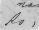Ro,
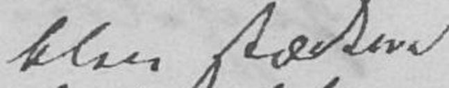blev stærkere
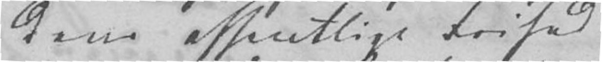dens offentlige Frihed
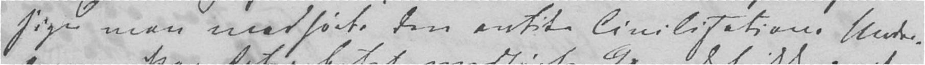siger man medførte den antike Civilisations Under-
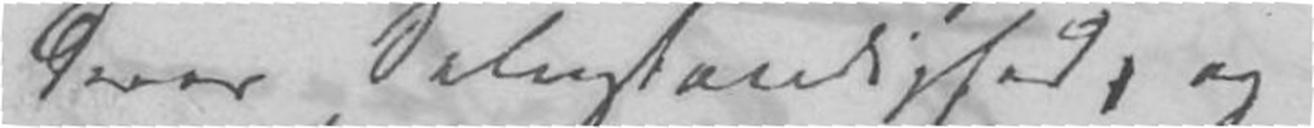deres Selvstændighed, og
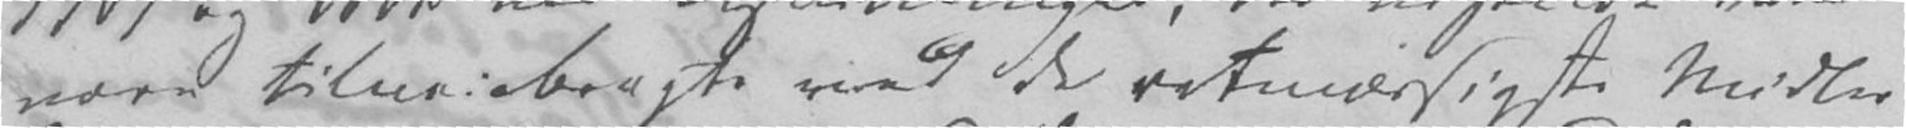vare tilveiebragte ved de retmæssigste Midler
